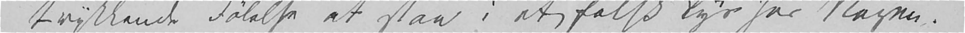trykkende Følelse at staa i et falsk Lys for Nogen.
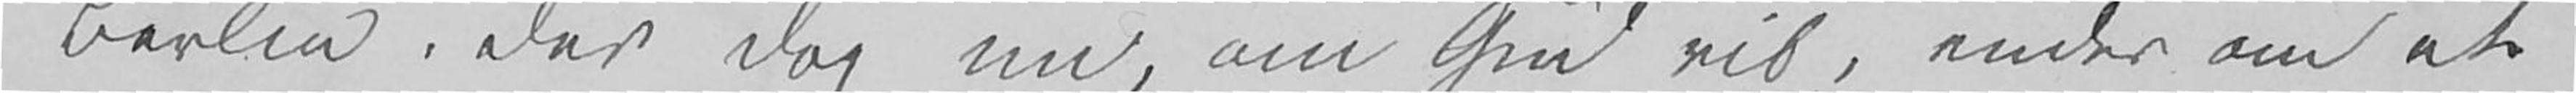i Berlin, der dog nu, om Gud vil, ender om et
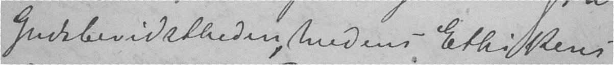Gudsbevidstheden, medens Ethikens
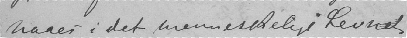naaes i det menneskelige Levnet.
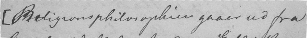[Religionsphilosophien gaaer ud fra
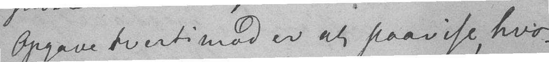Opgave tvertimod er at paavise, hvor-
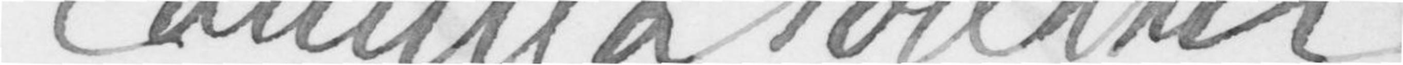Camilla Collett
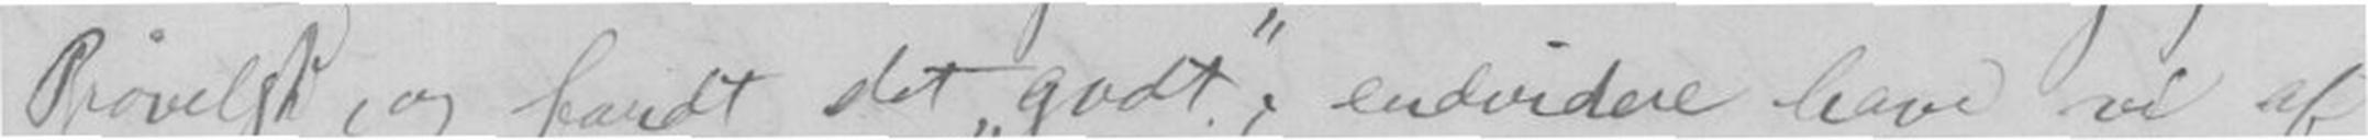Prøvelse, og fandt det godt, endvidere have vi af
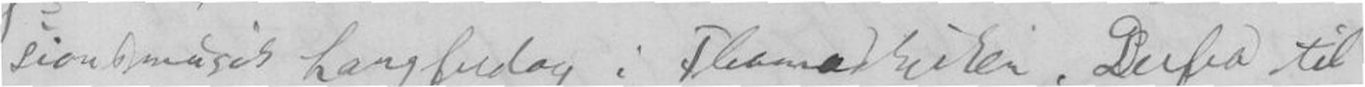sionsmusik Langfredag i Thomaskirken. Derfra til
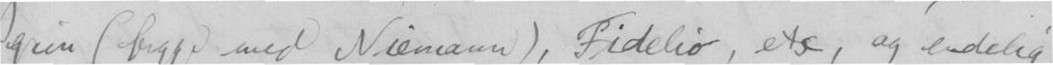grim (begge med Niemann), Fidelio, etc, og endelig
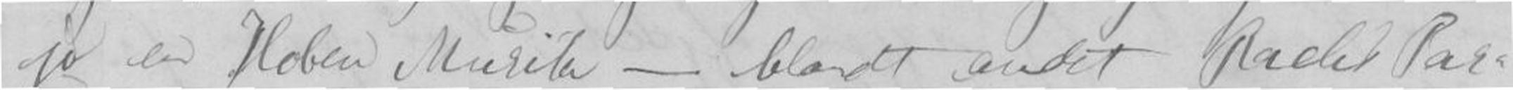jo en Hoben Musik - blandt andet Bachs Pas-
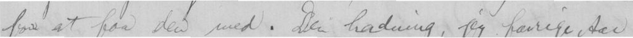for at faa den med. Den hadning, jeg forige Aar
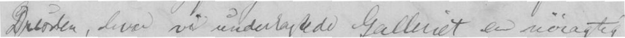Dresden, hvor vi underkastede Galleriet en nøiagtig
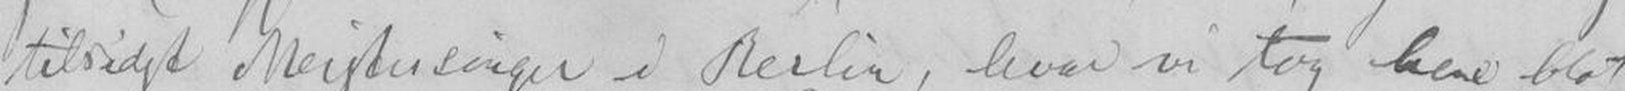tilsidst Mestersänger i Berlin, hvor vi tog hen blot
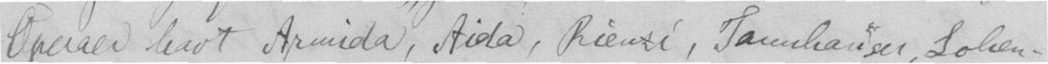Operaer havt Armida, Aida, Rienzi, Samihauser, Lohen-
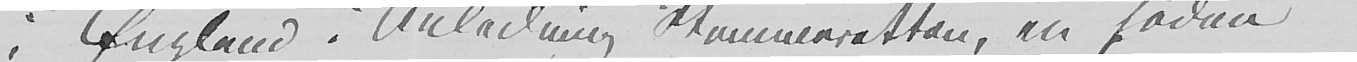i England i Anledning Stemmeretten, en sådan
